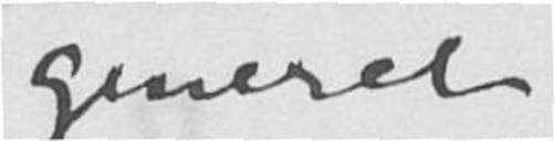generel
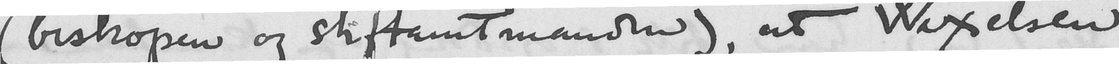(biskopen og stiftamtmanden), at Wexelsen
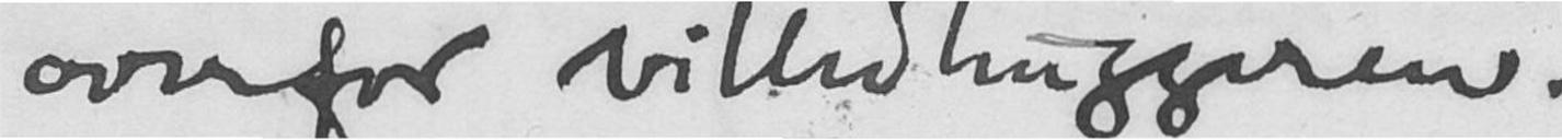overfor billedhuggeren.
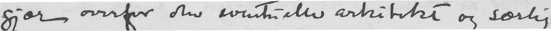gjær overfor den eventuelle arkitekt og særlig
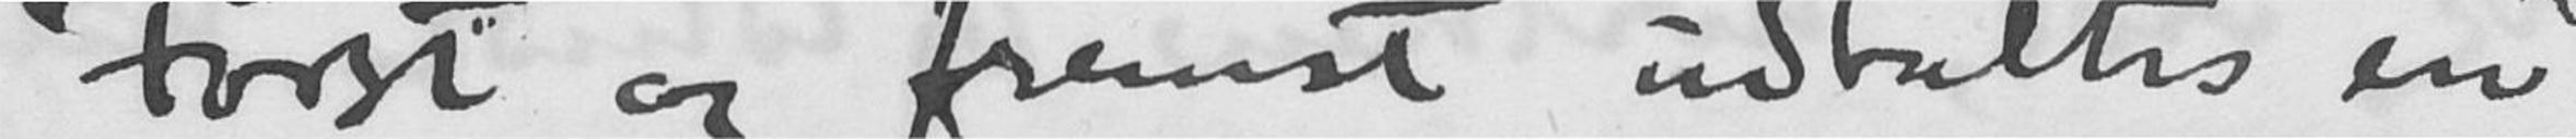Først og fremst udtaltes en
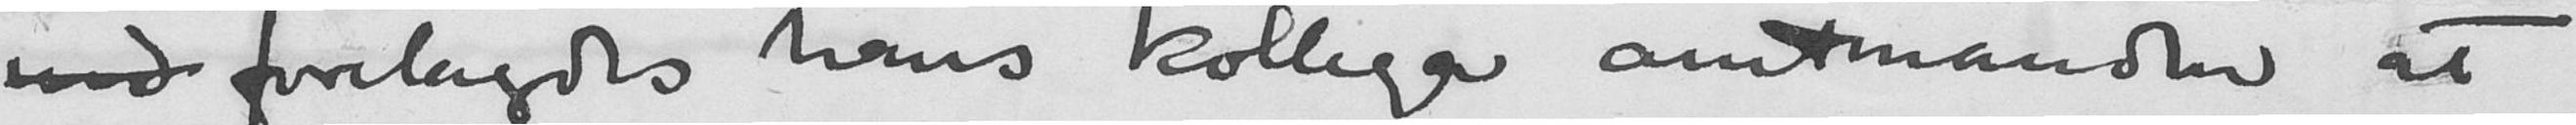med forelagdes hans kollega amtmanden at
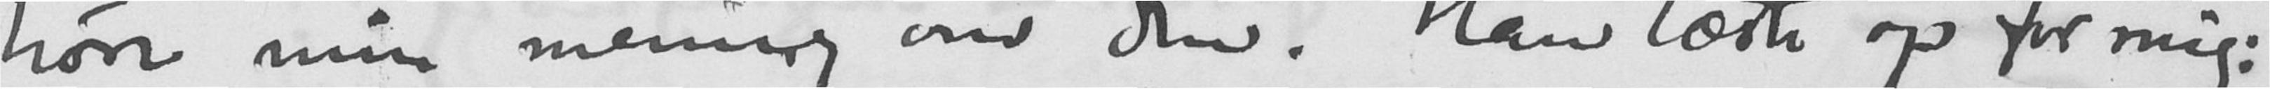høre min mening om den. Han læste op for mig:
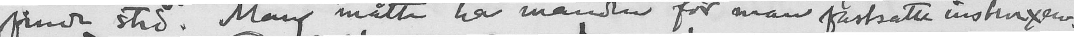finde sted. Man måtte ha manden før man fastsatte instruxen.
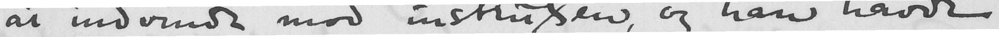at indvende mod instruxen, og han havde
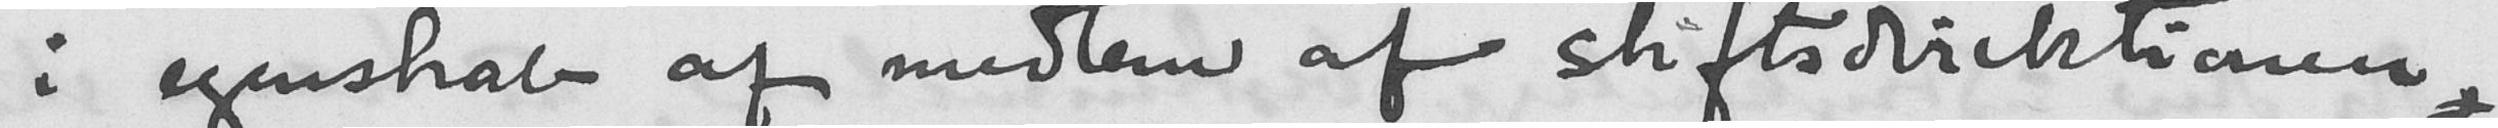i egenskab af medlem af stiftsdiretionen,
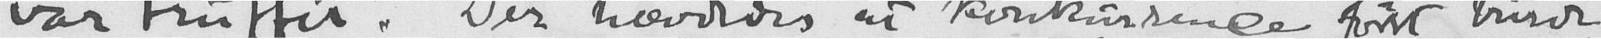var truffet. Der hævdedes at konkurrence først burde
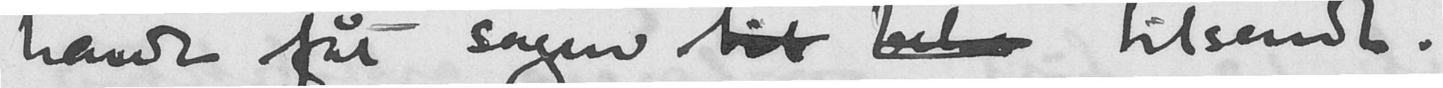havde fåt sagen til belas tilsendt.
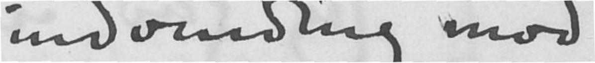indvending mod
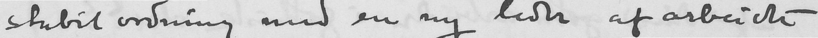stabil ordning med en ny leder af arbeidet
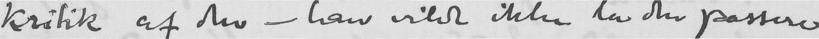kritik af den - han vilde ikke la den passere
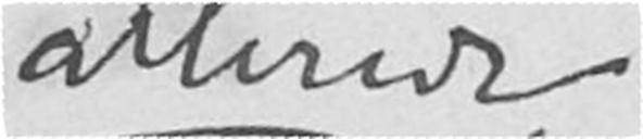allerede
